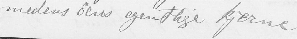medens øens egentlige kjerne
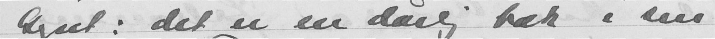Gynt: det er en dårlig bok i sin
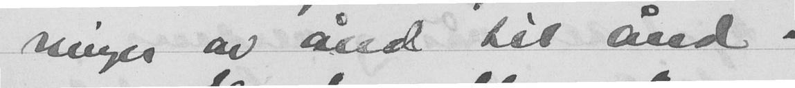ninger av ånd til ånd.
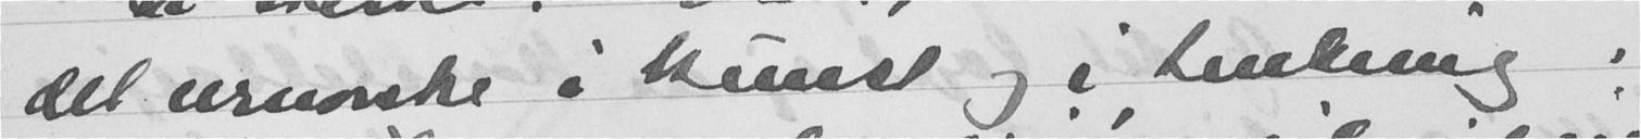det urnorske i kunst og i tenking.
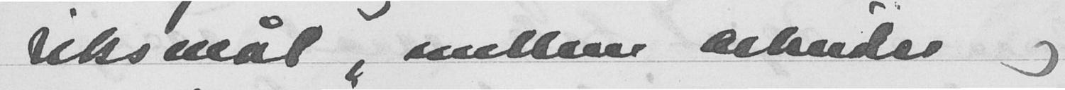riksmål, mellom arbeider og
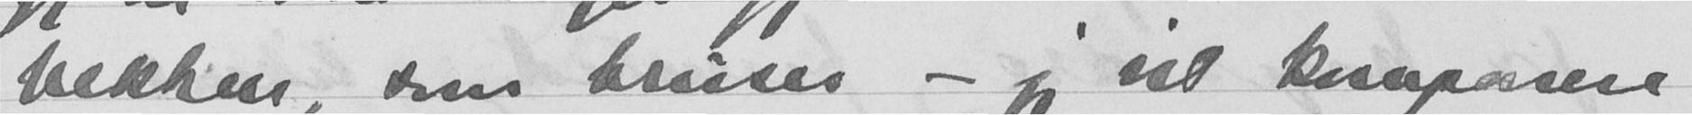bekken, som bruser - jeg vil komponere
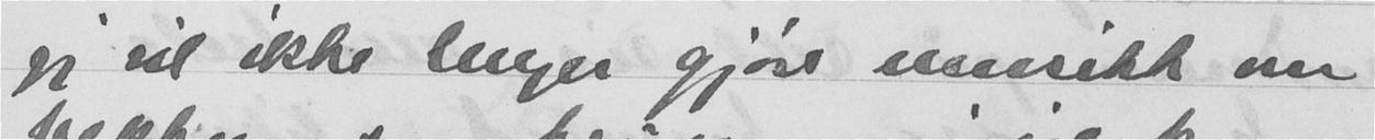jeg vil ikke lenger gjøre musikk om
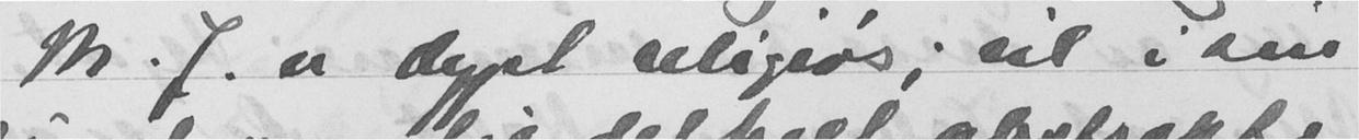M. J. er dypt religiøs; vil i sin
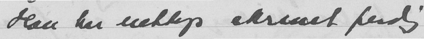Han har nettop skrevet ferdig
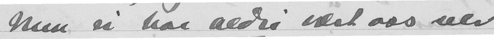Men vi har aldri vært oss selv
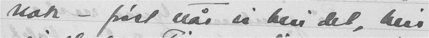nok - først når vi blir det, blir
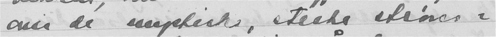om de supteske, sterke strøm-
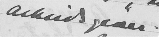arbeidsgiver.
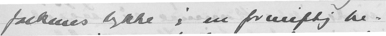folkenes lykke i en fremtidig be-
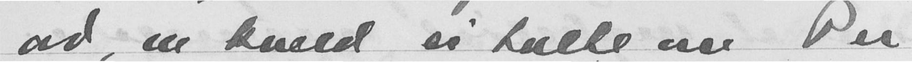ord, en kveld vi talte om Per
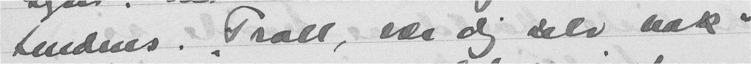tendens: "Troll, vær dig selv nok"
